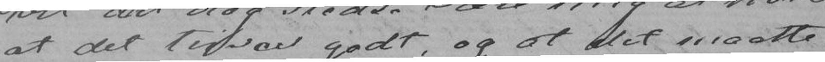at det trives godt, og at det maatte
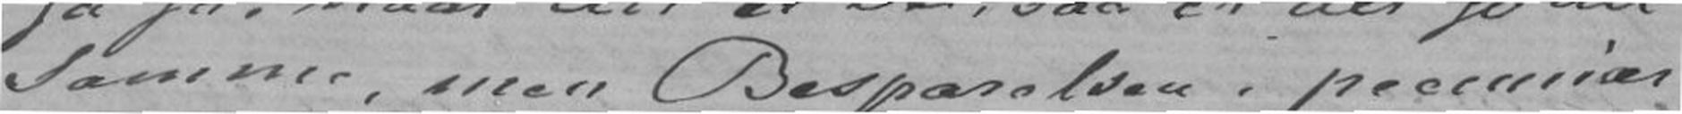Samme, men Besparelsen i pecuniær
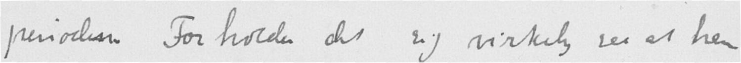perioden. Forholder det sig virkelig saa at han
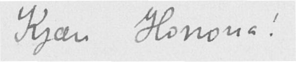Kjære Honoria!
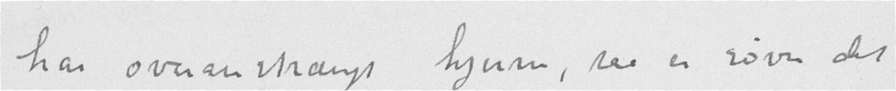har overanstrængt hjerne, saa er søvn det
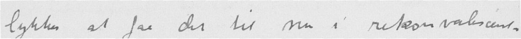lykkes at faa det til nu i rekonvalescent-
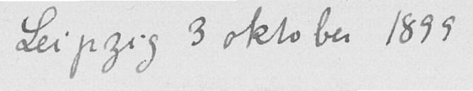Leipzig 3 oktober 1899
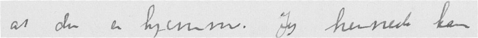at du er hjemme. Jeg hernede kan
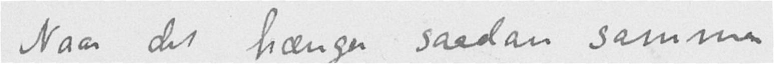Naar det hænger saadan sammen
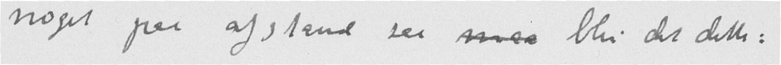noget paa afstand saa maa blir det dette:
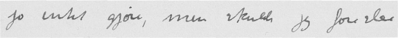jo intet gjøre, men skulde jeg foreslaa
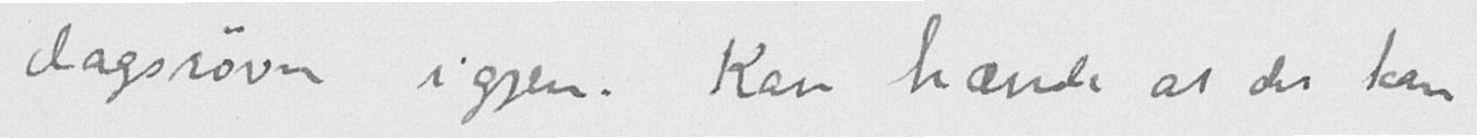dagssøvn igjen. Kan hænde at du kan
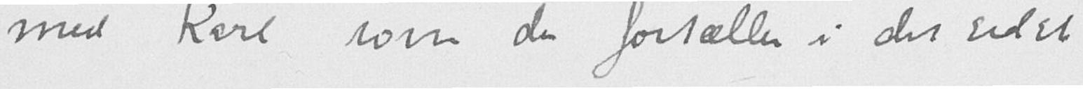med Karl som du fortæller i det sidste
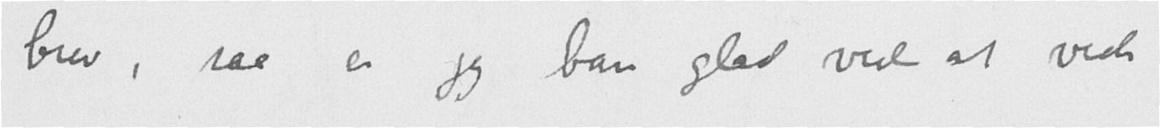brev, saa er jeg bare glad ved at vide
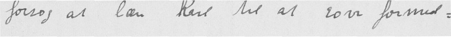forsøg at lære Karl til at sove formid-
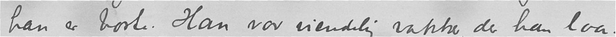han er borte. Han var uendelig vakker, der han laa.
